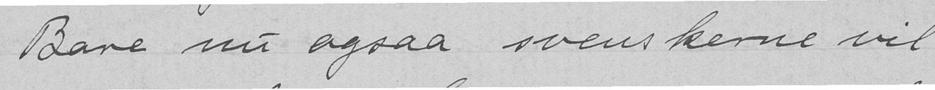Bare nu ogsaa svenskerne vil
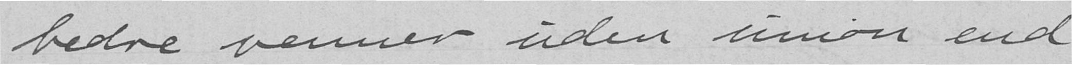bedre venner uden union end
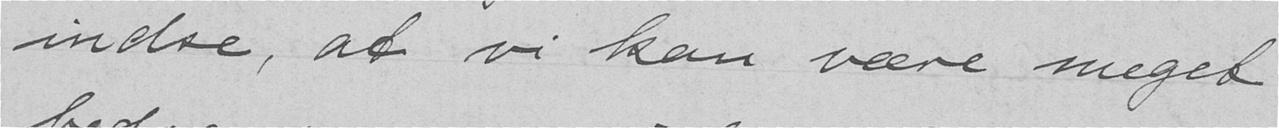indse, at vi kan være meget
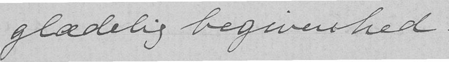glædelig begivenhed.
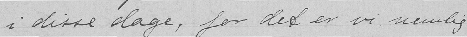i disse dage, for det er vi nemlig
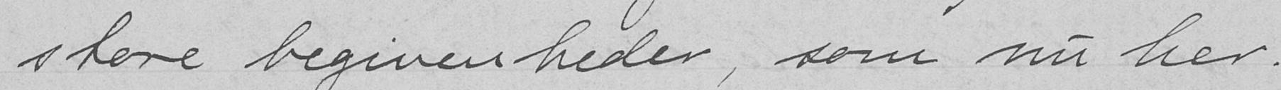store begivenheder, som nu her.
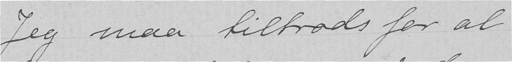Jeg maa tiltrods for al
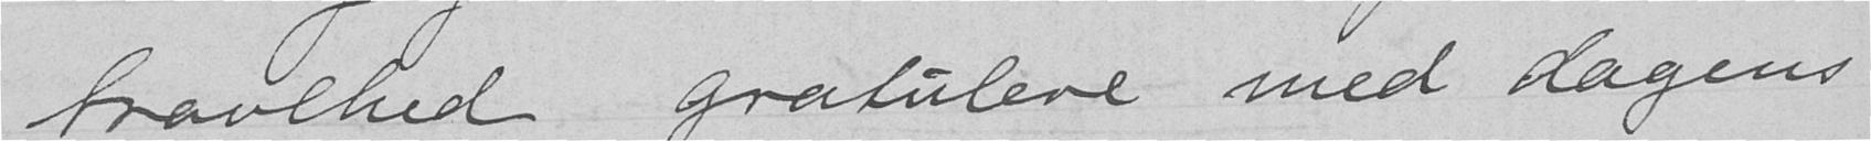travlhed gratulere med dagens
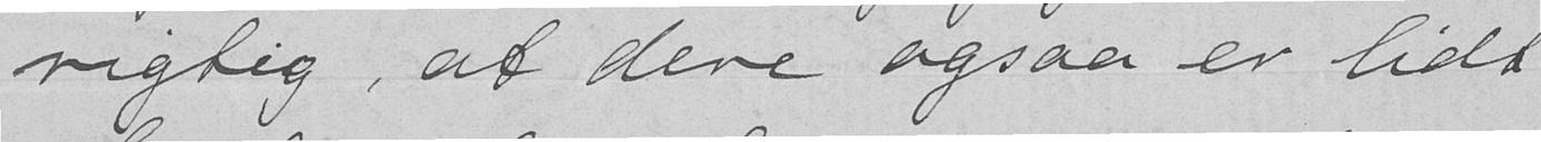rigtig, at dere ogsaa er lidt
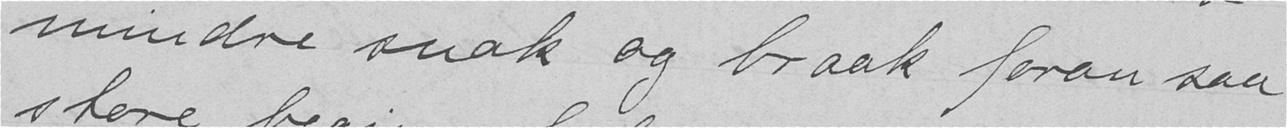mindre snak og braak foran saa
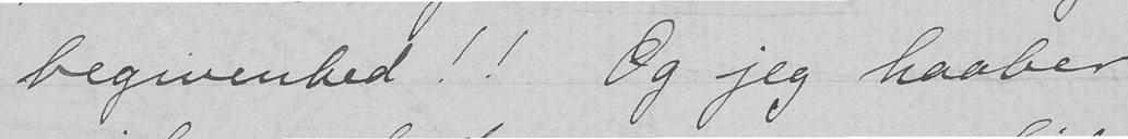begivenhed!! Og jeg haaber
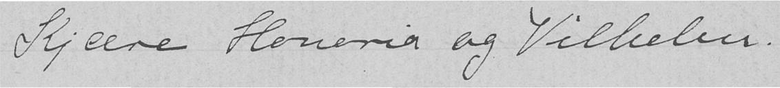Kjære Honoria og Vilhelm!
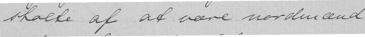stolte af at være nordmænd
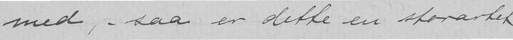med, - saa er dette en storartet
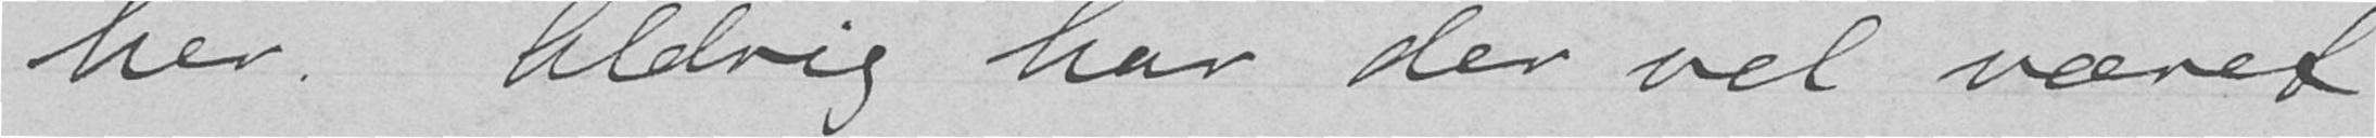her. Aldrig har der vel været
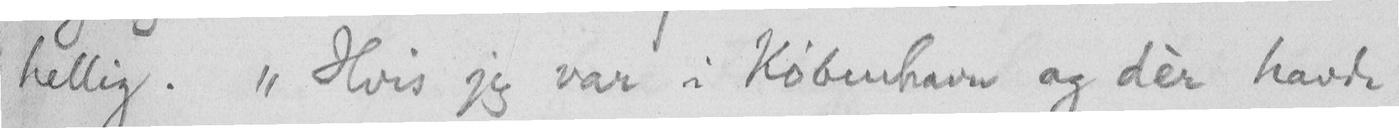hellig. "Hvis jeg var i København og dèr havde
